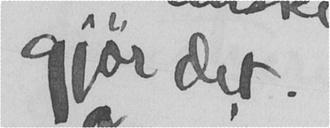gjør det.
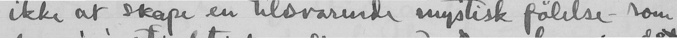ikke at skape en tilsvarende mystisk følelse som
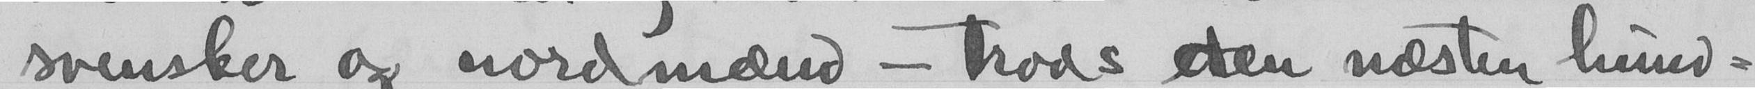svensker og nordmænd - trods den næsten hund-
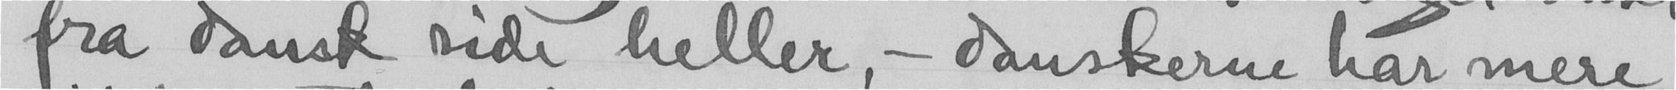fra dansk side heller, - danskerne har mere
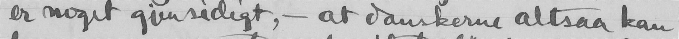er noget gjensidigt, - at danskerne altsaa kan
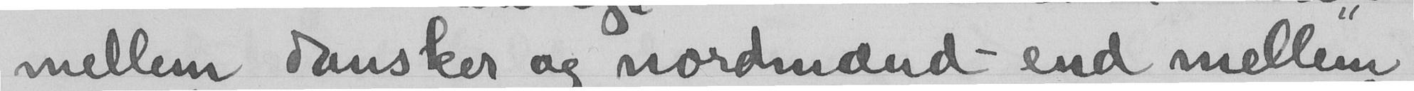mellem dansker og nordmænd - end mellem
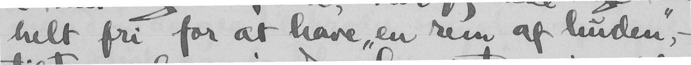helt fri for at have "en sem af huden",-
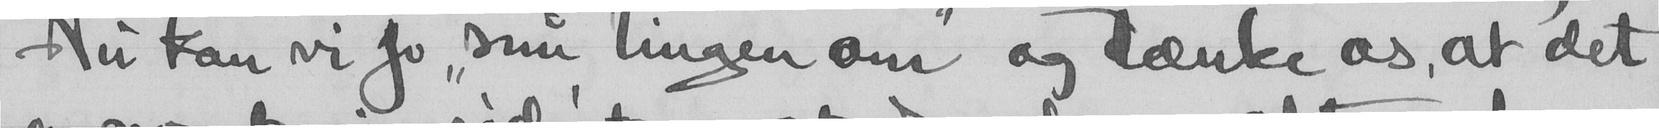Nu kan vi jo "snu tingen om og tænke os, at det
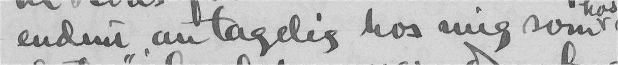endnu antagelig hos mig som
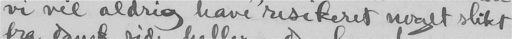vi vel aldrig have risikeret noget slikt
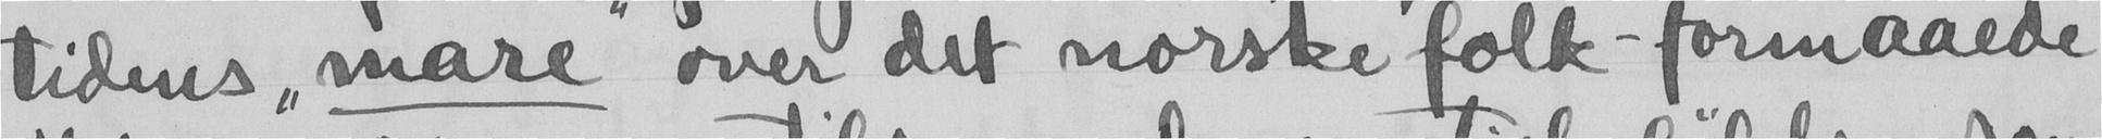tidens "mare" over det norske folk - formaaede
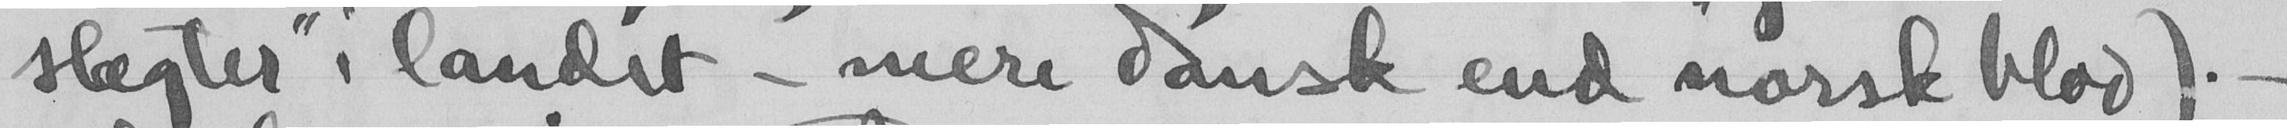slægter" i landet - mere dansk end norsk blod). -
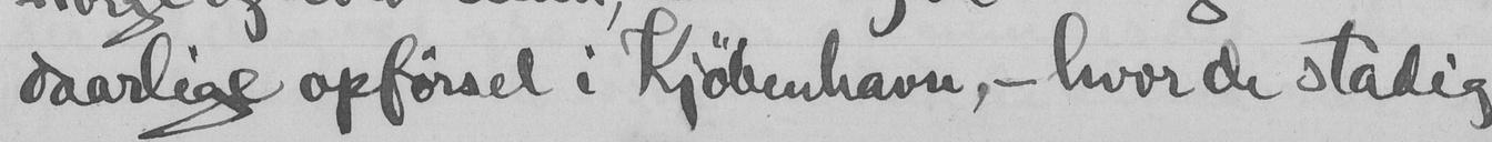daarlige opførsel i Kjøbenhavn, - hvor de stadig
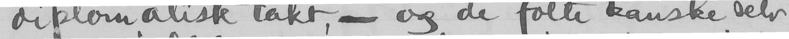deplomatisk takt, - og de følte kanske selv
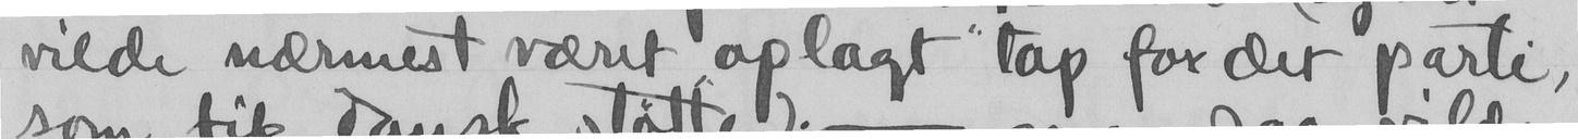vilde nærmest været oplagt tap for det parti,
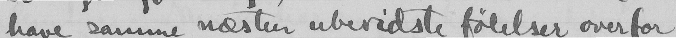have samme næsten ubevidste følelser overfor
